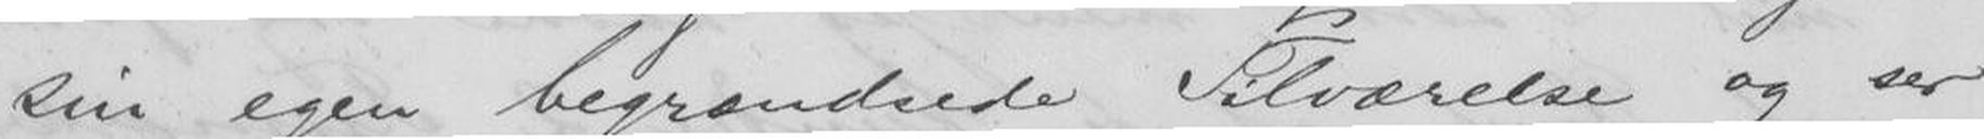sin egen begrændsede Tilværelse og ser
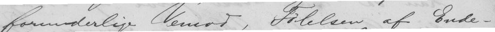forunderlige Vemod, Følelsen af Ende-
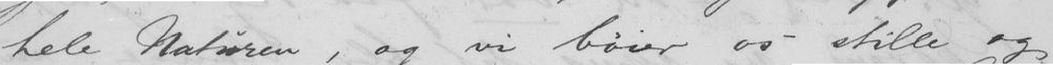hele Naturen, og vi bøier os stille og
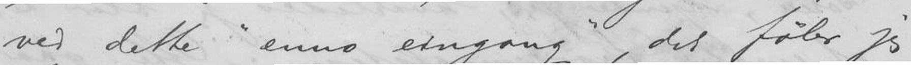ved dette "enno engong", det føler jeg
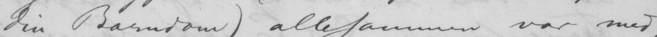din Barndom) allesmmen var med,
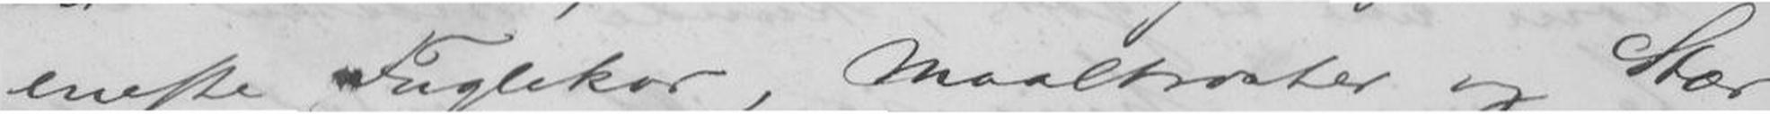eneste Fuglekor, Maaltroster og Stær
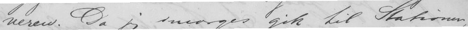veren. Da jeg imorges gik til Stationen,
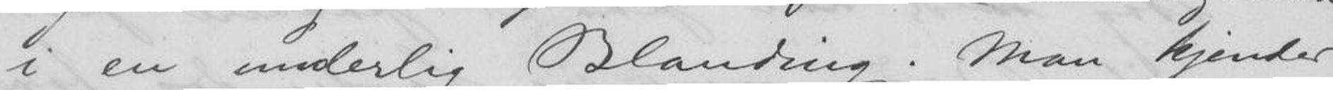i en underlig Blanding. Man kjender
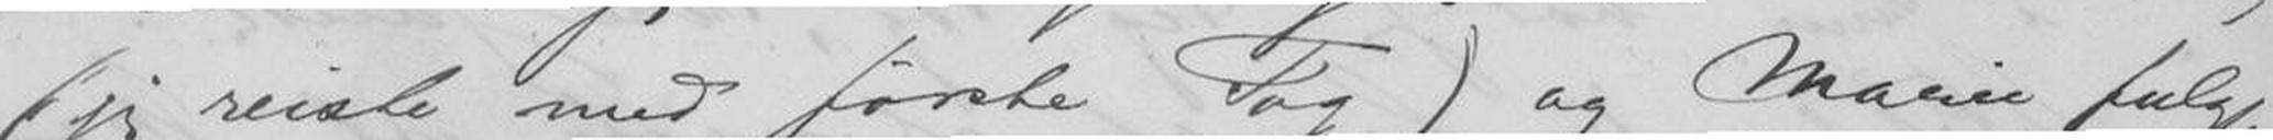(jeg reiste med første Tog) og Marie fulgte
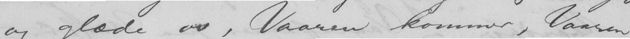og glæde os, Vaaren kommer, Vaaren
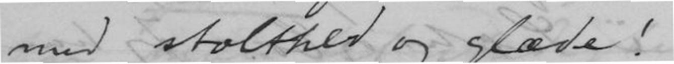med stolthed og glæde!
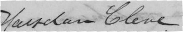Halfdan Cleve
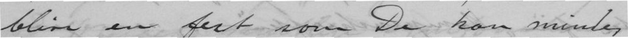blive en fest som De kan mindes
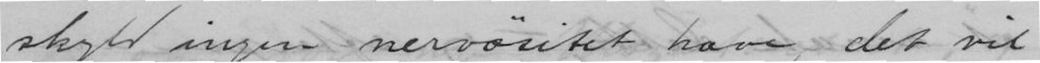skyld ingen nervøsitet have, det vil
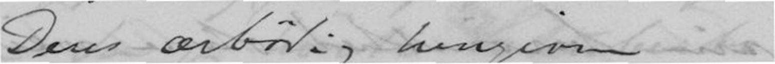Deres ærbødig hengivne
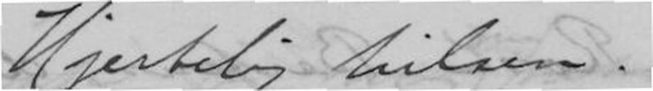Hjertelig hilsen.
